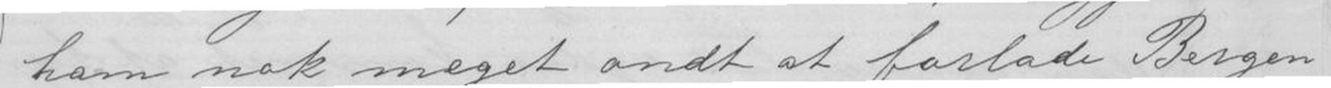ham nok meget ondt st forlade Bergen
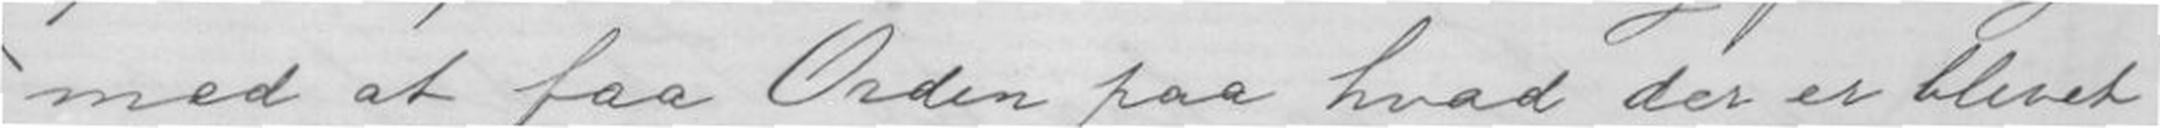med at faa Orden paa hvad der er blevet
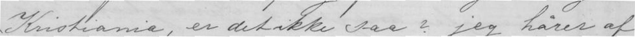Kristiania, er det ikke saa? jeg hører af
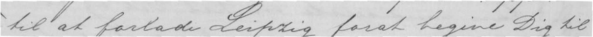til at forlade Leipzig forat begive Dig til
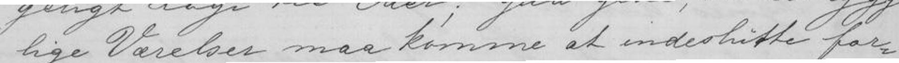lige Værelser maa komme at indeslutte for-
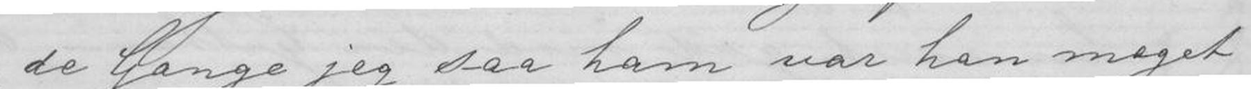de Gange jeg saa ham var han meget
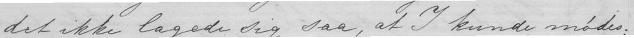det ikke lagede sig saa, at I kunde mødes;
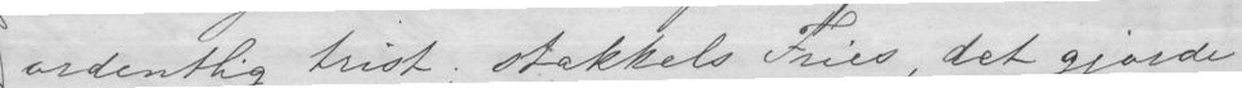ordentlig trist, stakkels Fries, det gjorde
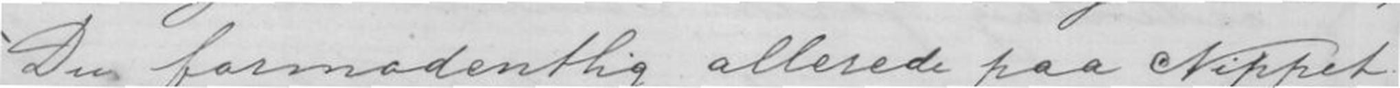Du formodentlig allerede paa Nippet
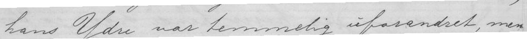hans Ydre var tennelig uforandret, men
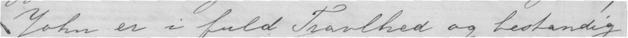John er i fuld Travlhed og bestandig
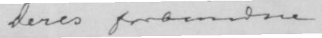Deres forbundne
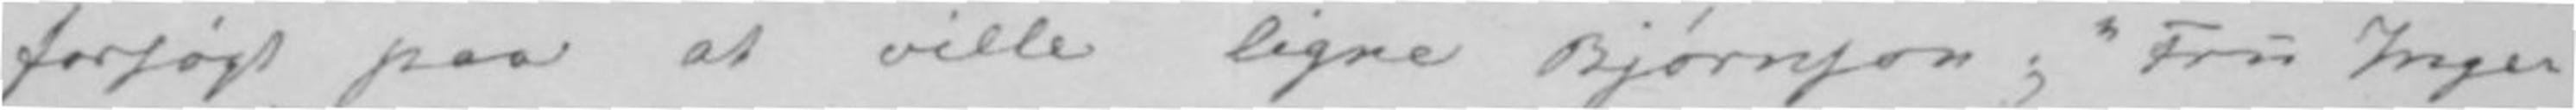forsøgt paa at ville ligne Bjørnson; «Fru Inger
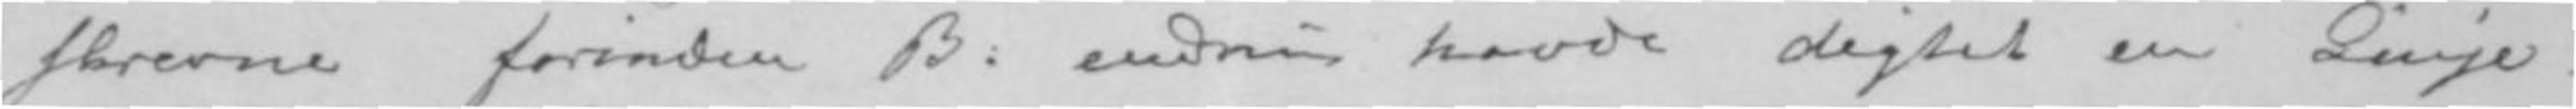skrevne forinden B: endnu havde digtet en Linje.
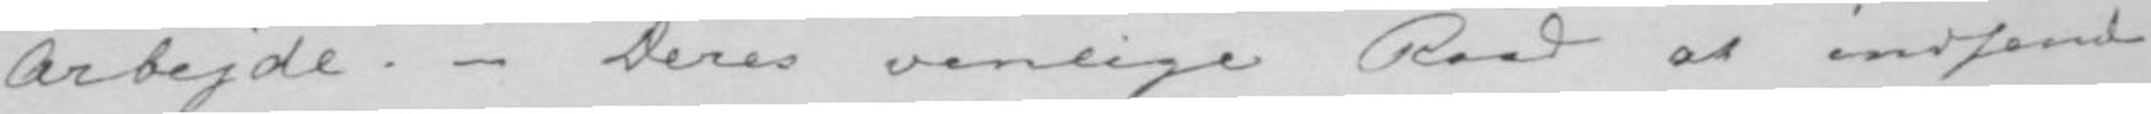Arbejde.- Deres venlige Raad at indsende
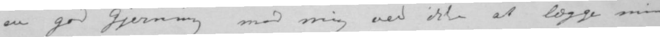en god Gjerning mod mig ved ikke at lægge min
